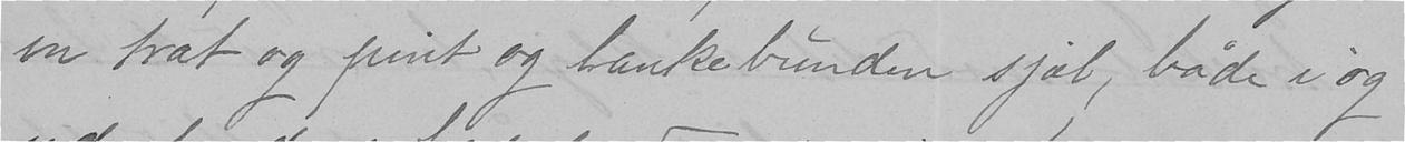en træt og pint og tankebunden sjæl, både i og
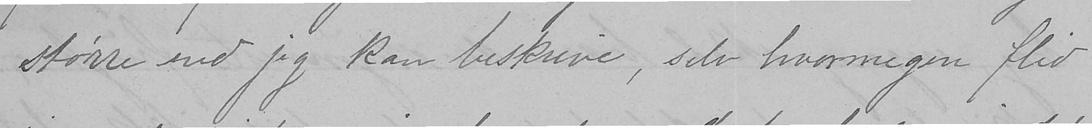større end jeg kan beskrive, selv hvormegen flid
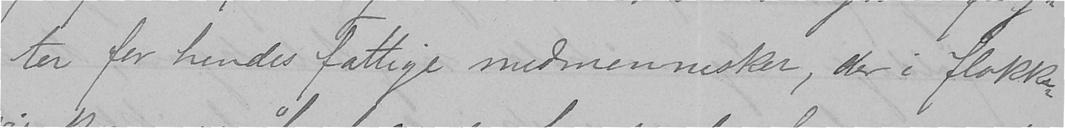ter for hendes fattige medmennesker, der i flokke-
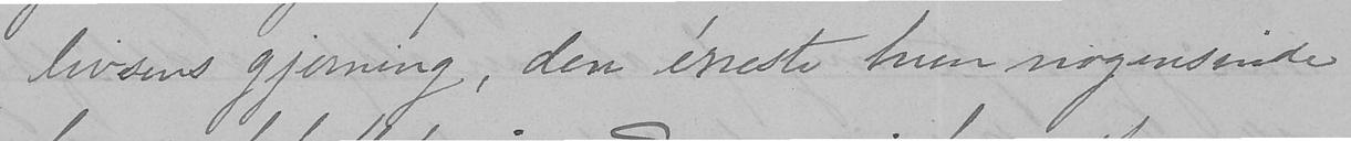livsens gjerning, den eneste hun nogensinde
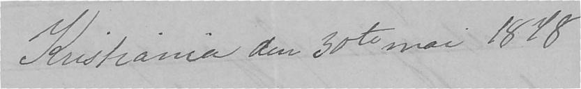Kristiania den 30te mai 1878
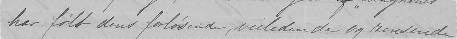har følt dens forløsende, veiledende og rensende
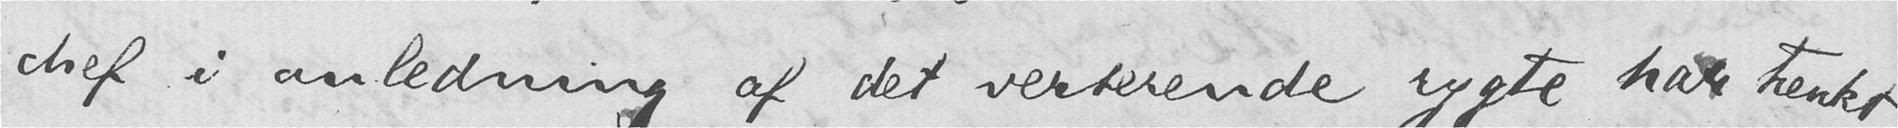chef i anledning af det verserende rygte har tænkt
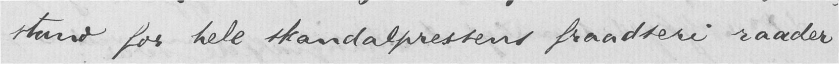stand for hele skandalpressens fraadseri raader
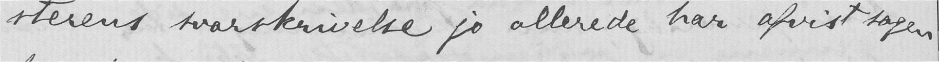sterens svarskrivelse jo allerede har afvist sagen
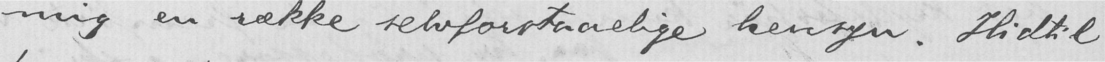mig en række selvforstaaelige hensyn. Hidtil
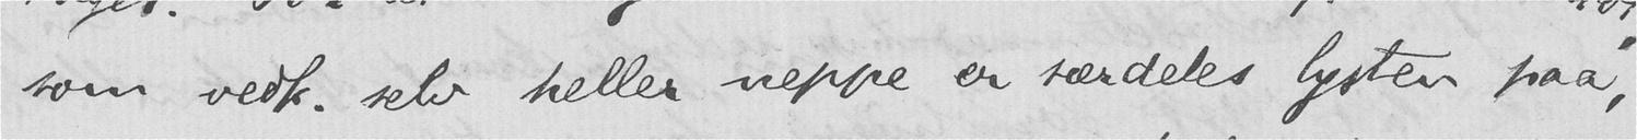som vedk. selv heller neppe er særdeles lysten paa,
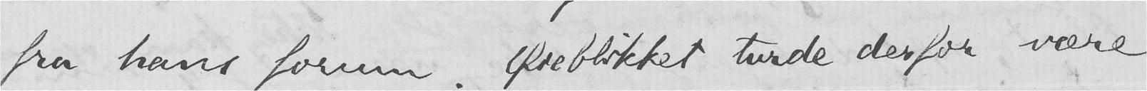fra hans forum. Øieblikket turde derfor være
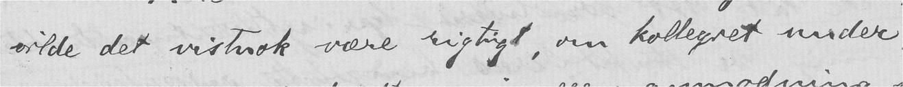vilde det vistnok være rigtigt, om kollegiet under-
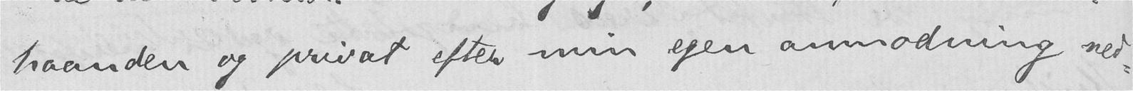haanden og privat efter min egen anmodning ned-
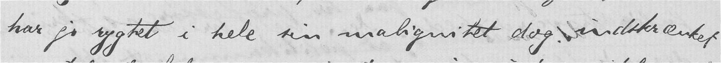har jo rygtet i hele sin malignitet dog indskrænket
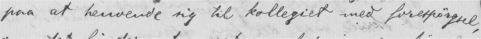paa at henvende sig til kollegiet med forespørgsel,
